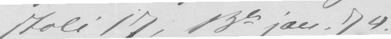stoli 17, 13de jan. 74
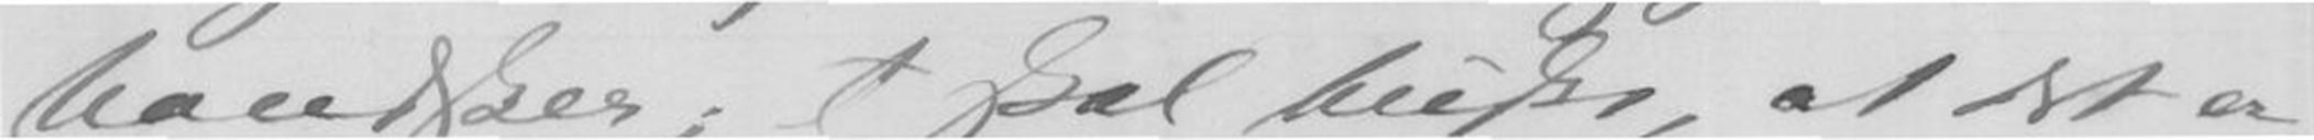handsker; I skal huske, at det er
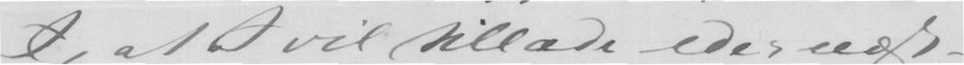I, at I vil tillade eder næst-
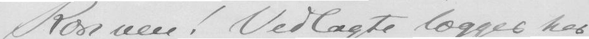Kære ven! Vedlagte lægges hos
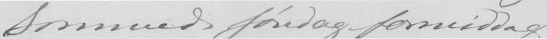kommende søndag formiddag
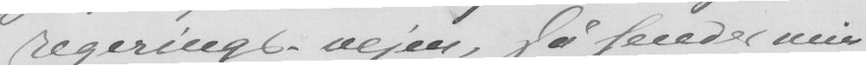regerings-vejen, så sendes min
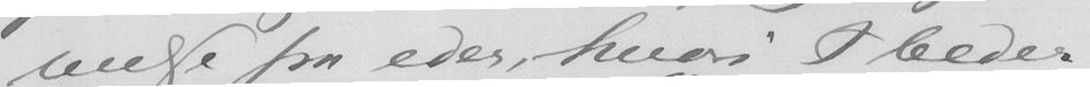velse fra eder hvori I beder
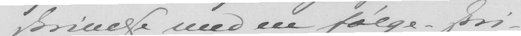skrivelse med en følge-skri-
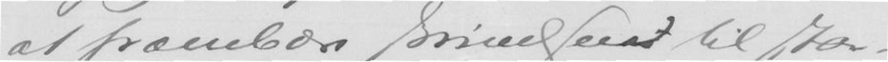at fræmbære skrivelsen til stor-
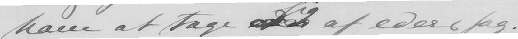ham at tage sig af eders sag.
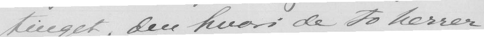tinget, den hvori de to herrer
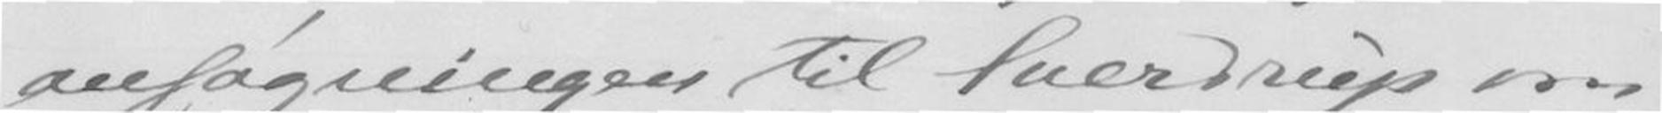ansøgningen til Sverdrup om
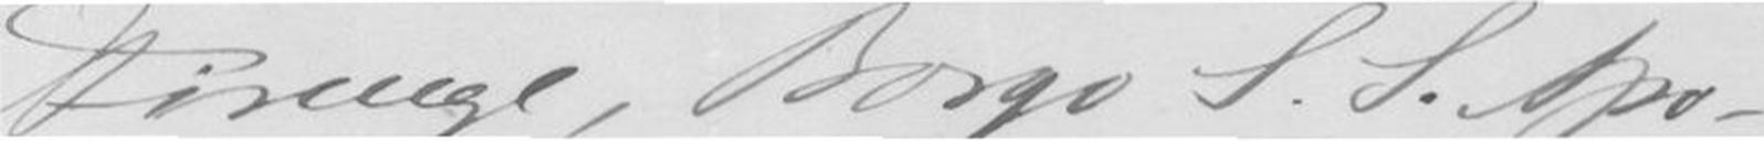Firenze, Borgo S.S. Apo-
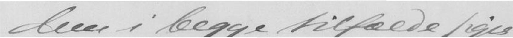Men i begge tilfælde siger
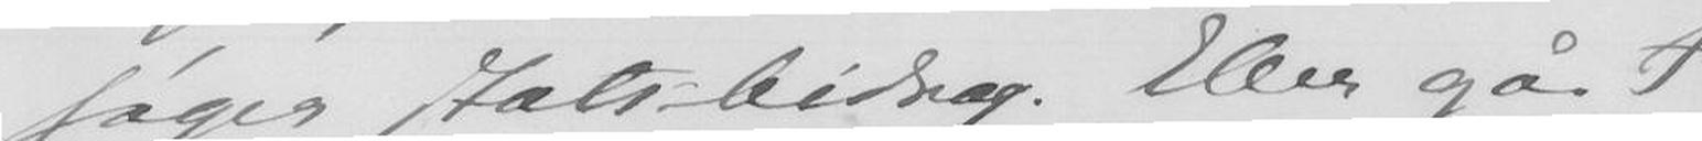søger statsbidrag. Eller går I
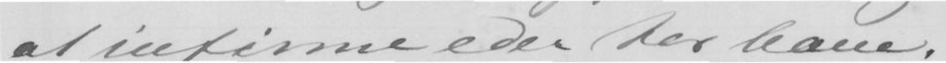at infinne eder hos ham.
